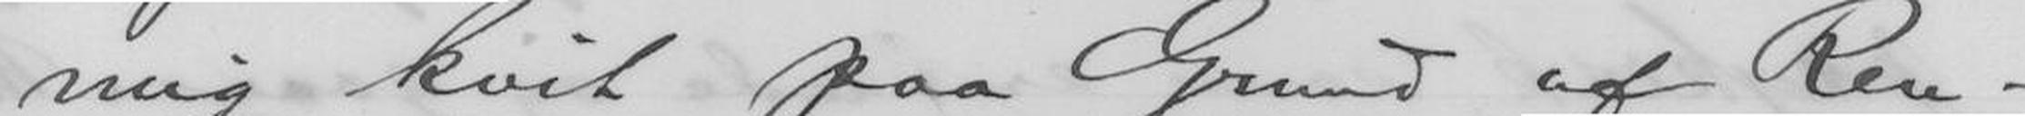mig kvit paa Grund af Ren-
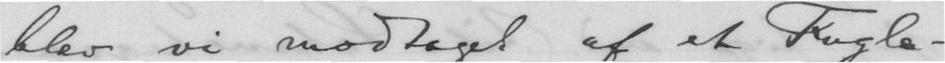blev vi modtaget af et Fugle-
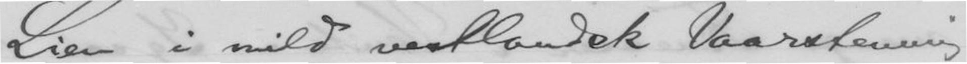Lien i mild vestlandsk Vaarstemning,
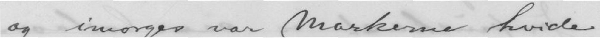og imorges var Markerne hvide
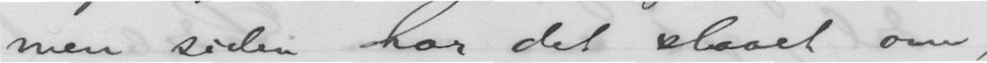men siden har det slaaet om,
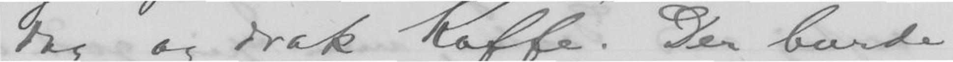dag og drak Kaffe. Der burde
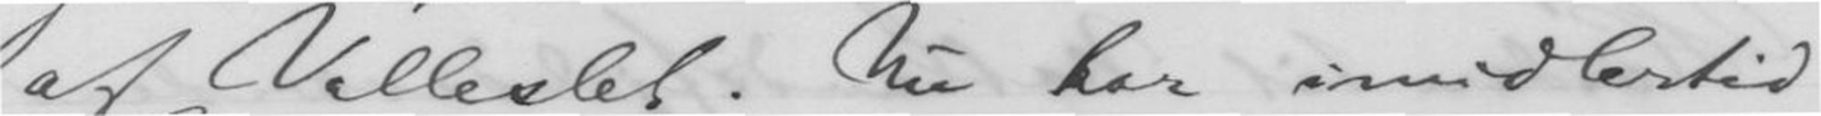af Valleslet. Nu har imidlertid
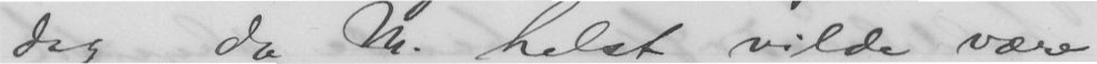dag, da M. helst vilde være
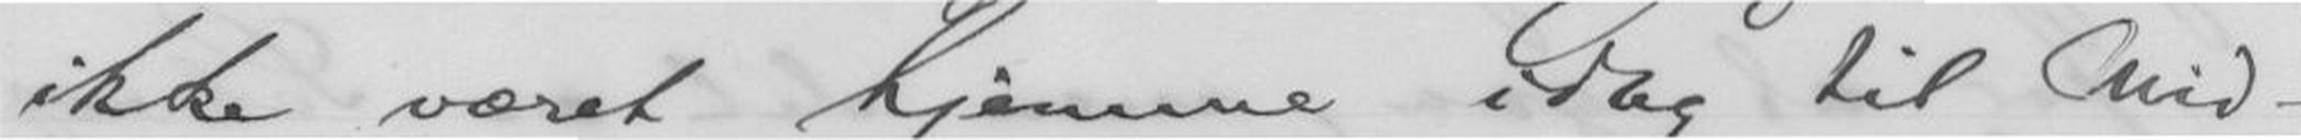ikke været hjemme idag til Mid-
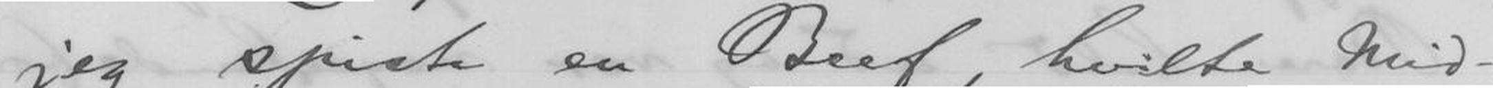jeg spiste en Beef, hvilte Mid-
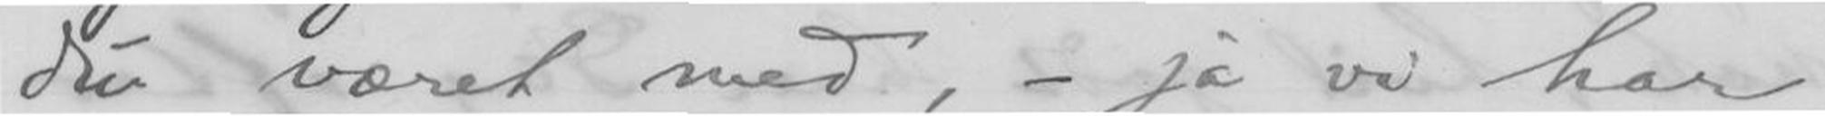Du været med, - ja vi har
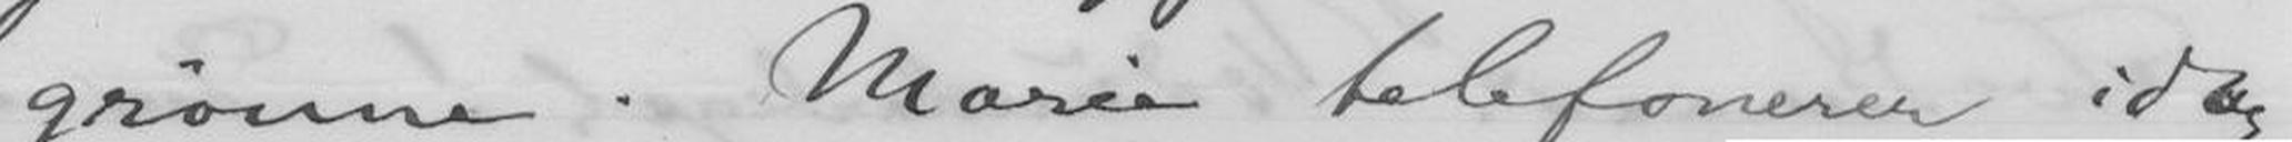grønne. Marie telefonerer idag,
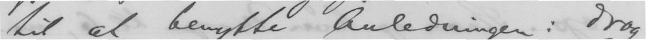til at benytte Anledningen: drog
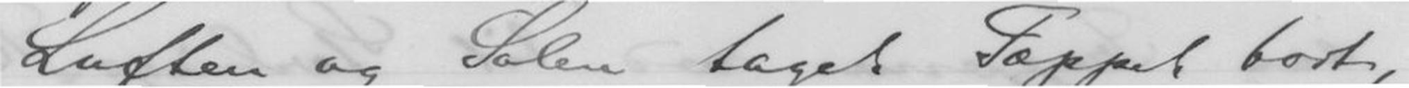Luften og Solen taget Tæppet bort,
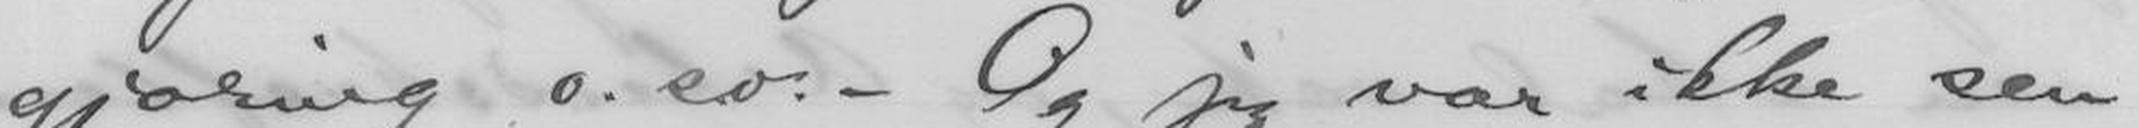gjøring o.s.v. - Og jeg var ikke sen
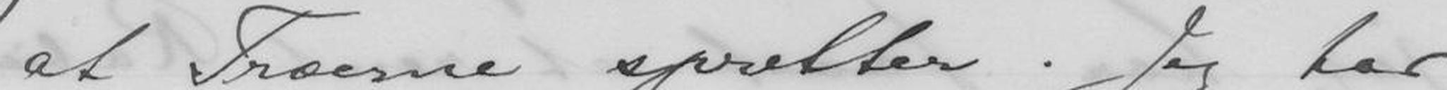at Træerne spretter. Jeg har
